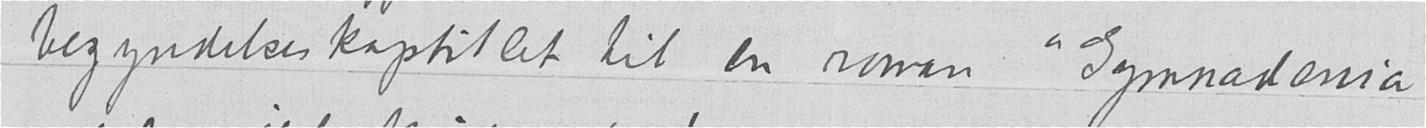begyndelseskapitlet til en roman "Gymnadenia"
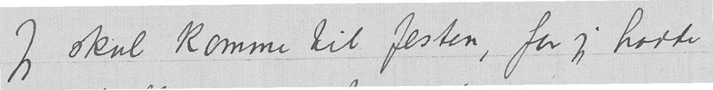Jeg skal komme til festen, for jeg hadde
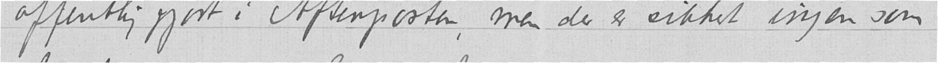offentliggjort i Aftenposten, men der er sikkert ingen som
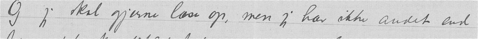Og jeg skal gjerne læse op, men jeg har ikke andet end
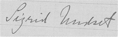Sigrid Undset
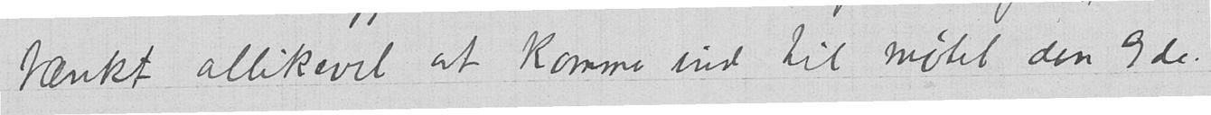tænkt allikevel at komme ind til møtet den 9de.
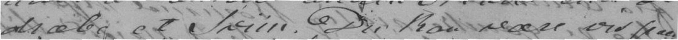dræbe et Sviin. Du kan være vis paa
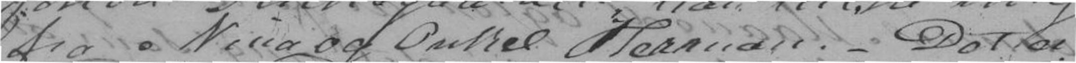fra Nina og Onkel Herman. - Det er
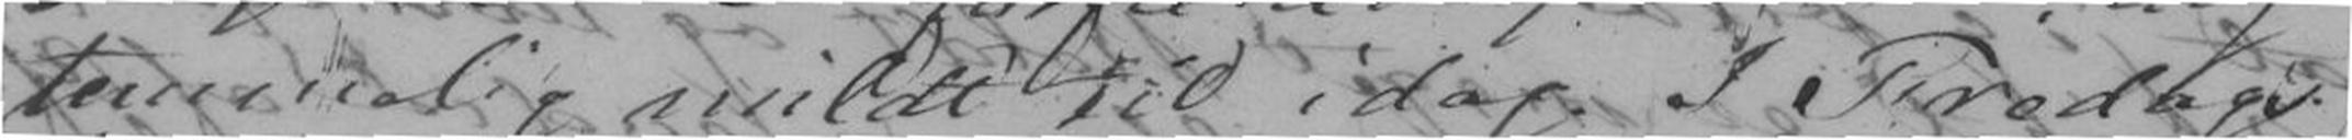temmelig mildt til idag. I Fredags
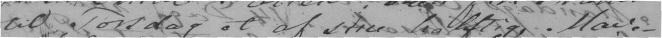til Torsdag et af sine heftige Mave-
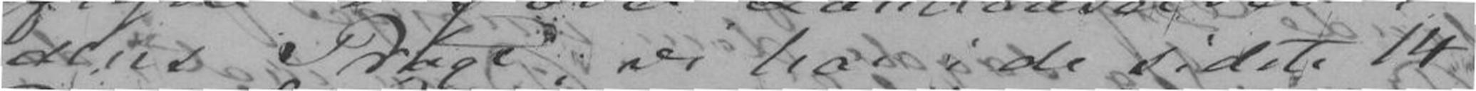dens Pragt; vi har i de sidste 14
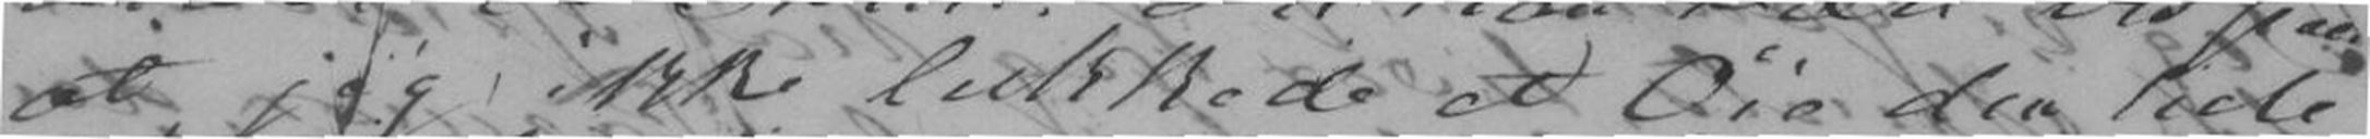at jeg ikke lukkede et Øie den hele
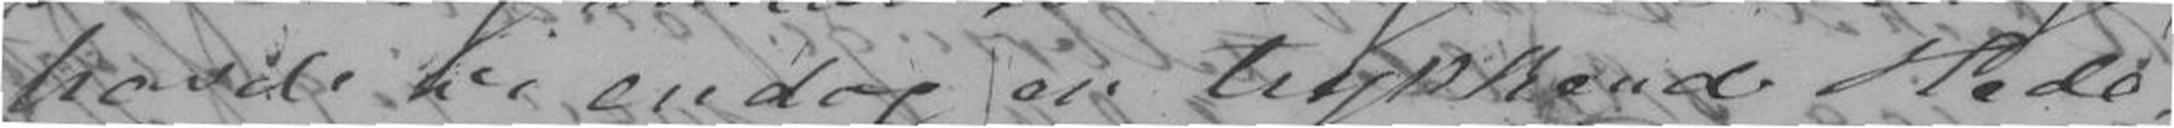havde vi endog en trykkende Hede,
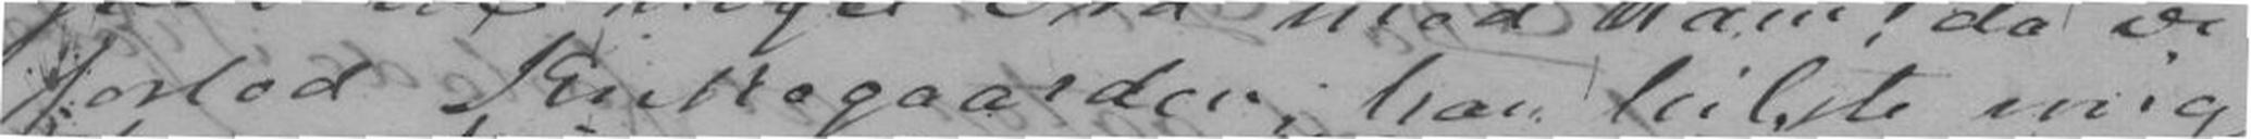forlod Kirkegaarden; han hilste mig
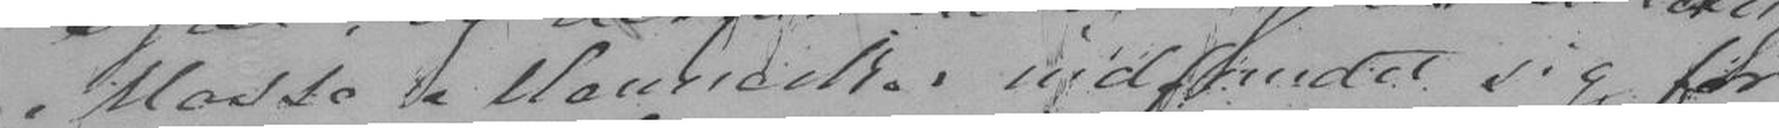Masse a Mennesker indfundet sig for
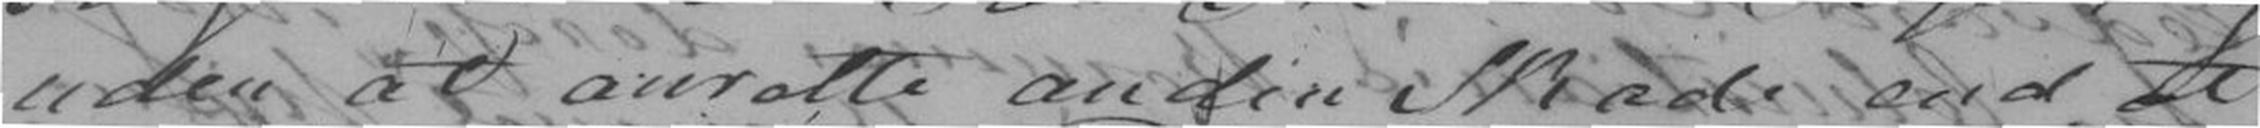uden at anrette anden Skade end at
